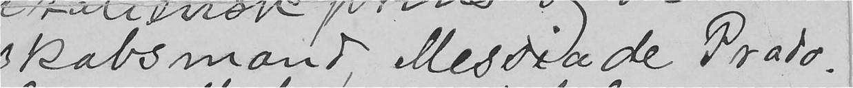skabsmand, Messia de Prado.
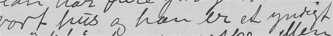vort hus å han er et yndigt
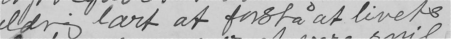aldrig lært at forstå at livets
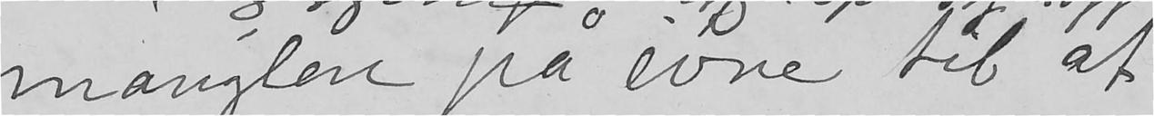manglen på evne til at
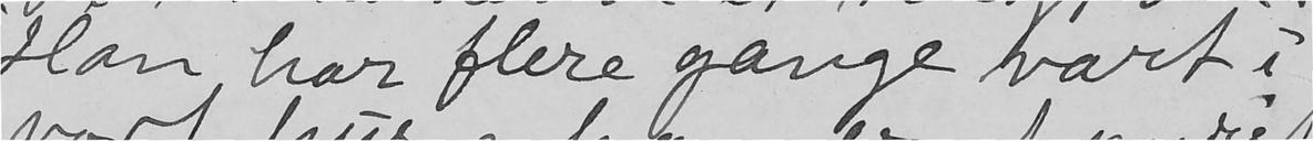Han har flere gange været i
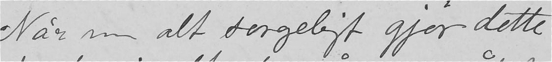Når nu alt sorgeligt gjør dette
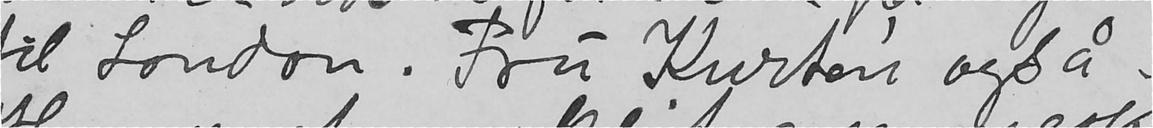til London. Fru Kurtén også.
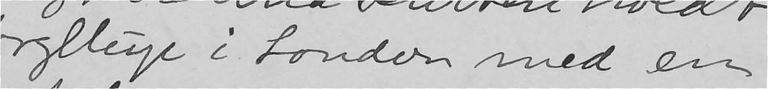bryllup i London med en
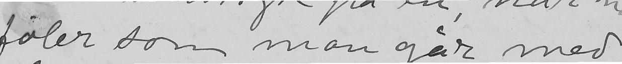føler som man går med
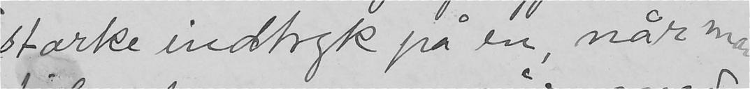stærke indtryk på en, når man
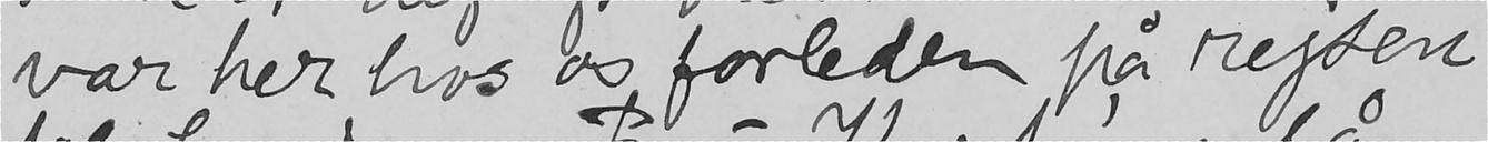var her hos og forleden på rejsen
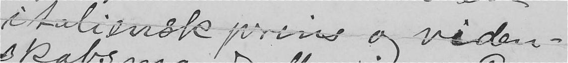italiensk prins og viden-
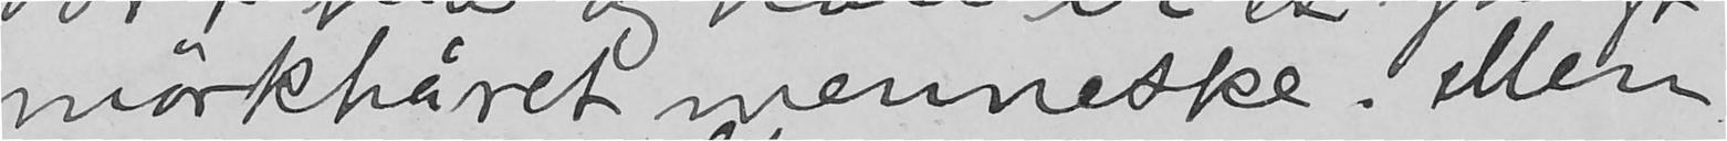mørkhåret menneske. Men
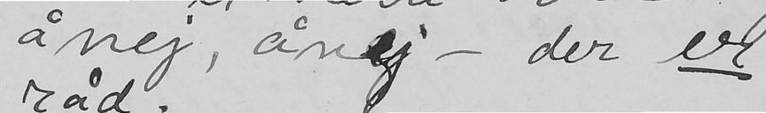- - ånej, ånej - der er
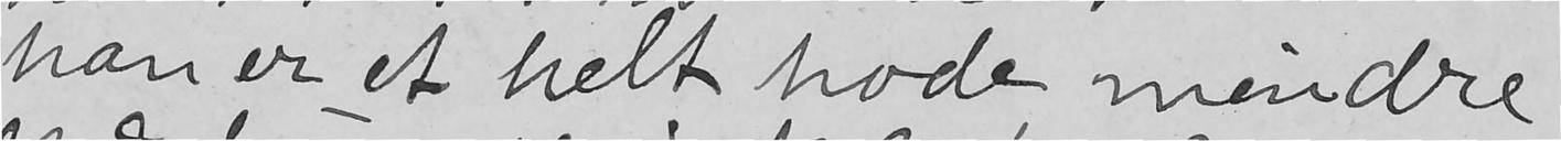han er et helt hode mindre
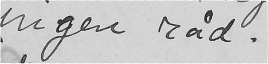ingen råd.
